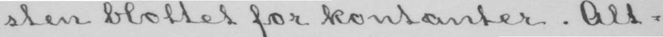sten blottet for kontanter. Alt-
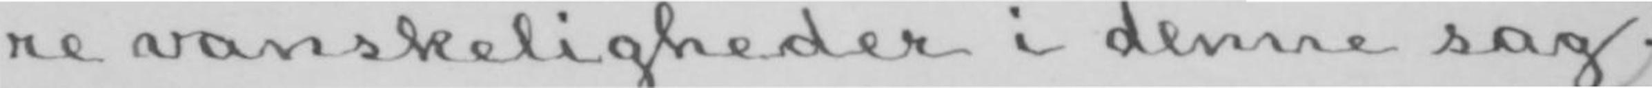re vanskeligheder i denne sag.
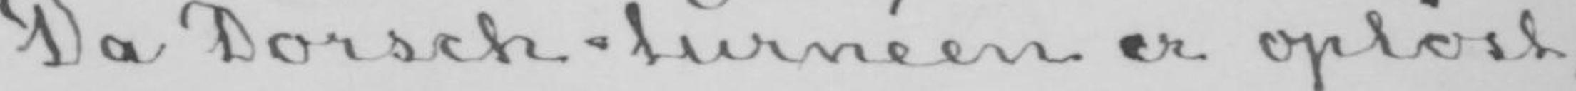Da Dorsch-turnéen er opløst,
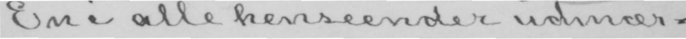En i alle henseender udmær-
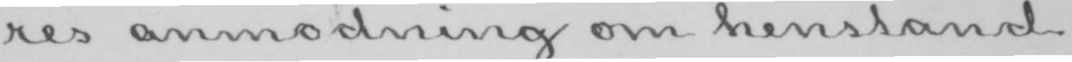res anmodning om henstand
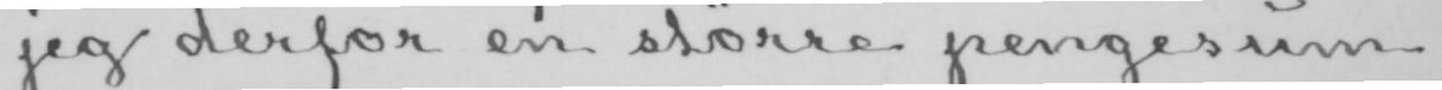jeg derfor en større pengesum
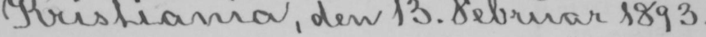Kristiania, den 13. Februar 1893.
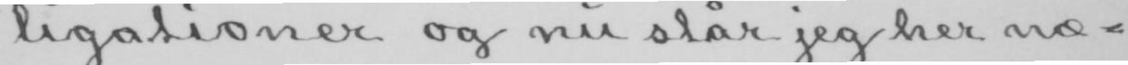ligationer og nu står jeg her næ-
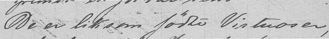De er liksom fødte Virtuoser,
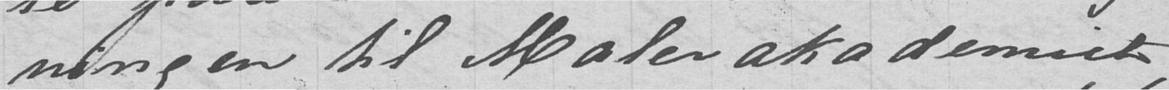ningen til Malerakademiet,
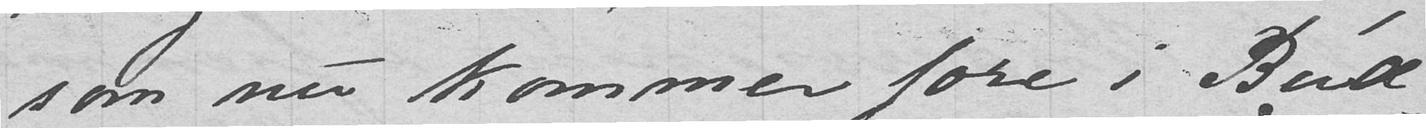som nu kommer fore i Bud-
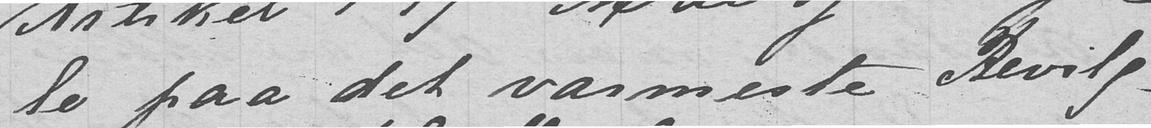le paa det varmeste Bevilg-
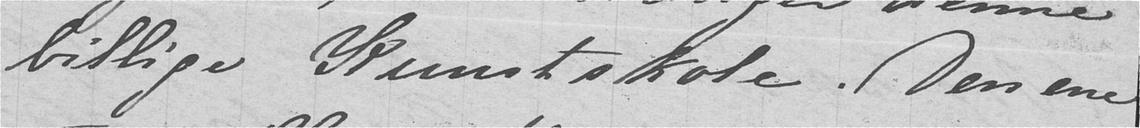billige Kunstskole. Den ene
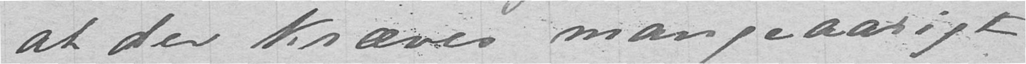at der Kræves mangeaarigt
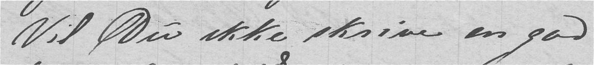Vil Du ikke skrive en god
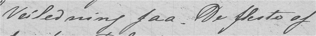Veiledning faa. De fleste af
